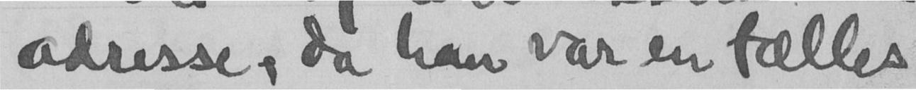adresse, da han var en fælles
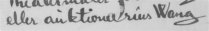eller auktionarius Wang
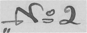No 2
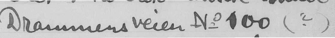Drammensveien No 100 (?)
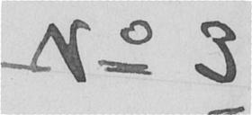No 3
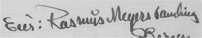Eier: Rasmus Meyers samling
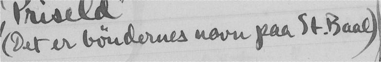(Det er bøndernes navn paa St. Baal)
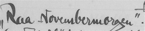"Raa Novembermorgen".
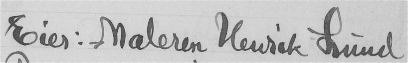Eier: Maleren Henrik Lund
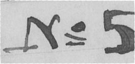No 5
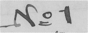No 1
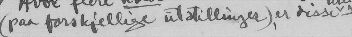(paa forskjellige utstillinger) er disse-
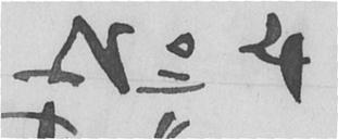No 4
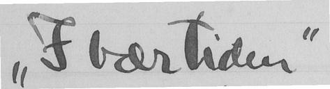"I bær tiden"
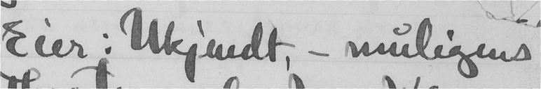Eier: Ukjendt, - muligens
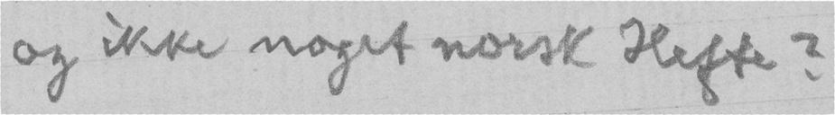og ikke noget norsk Hefte?
